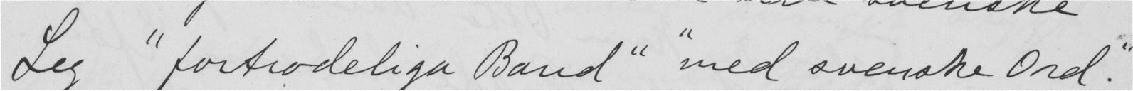Leg "fortrodeliga Band" "med svenske Ord".
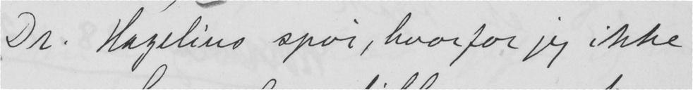Dr. Hagelius spør, hvorfor jeg ikke
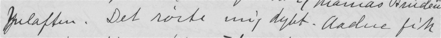Julaften. Det rørte mig dybt. Aadne fik
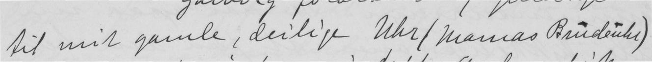til mit gamle, deilige Uhr (Mamas Brudeuhr)
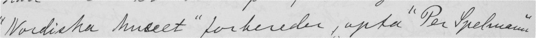"Nordiska Museet" forbereder, opta "Per Spelmann"
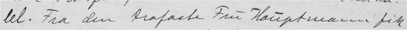lel. Fra den trofaste Fru Hauptmane fik
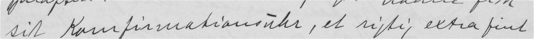sit Komfirmationsuhr, et rigtig extra fint
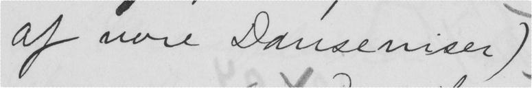af nore Danseviser)
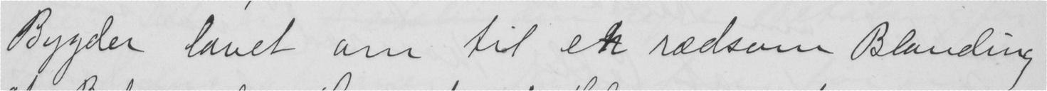Bygder lavet om til ek rædsom Blanding
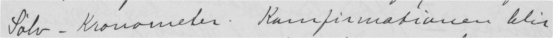Sølv- Kronometer. Komfirmationen blir
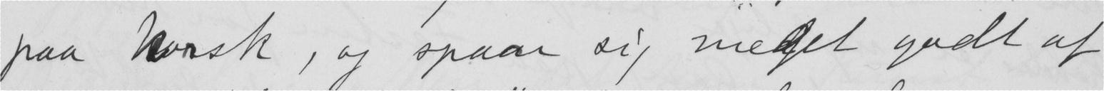paa Norsk, og spaar sig meget godt af
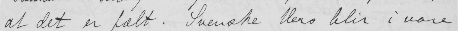at det er fælt. Svenske Vers blir i vore
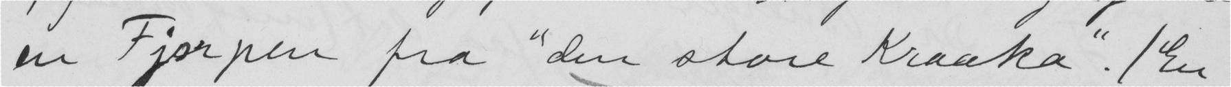en Fjærpen fra "den store Kraaka". (En
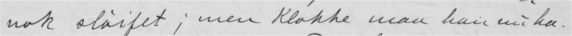nok sløifet; men Klokke maa han nu ha
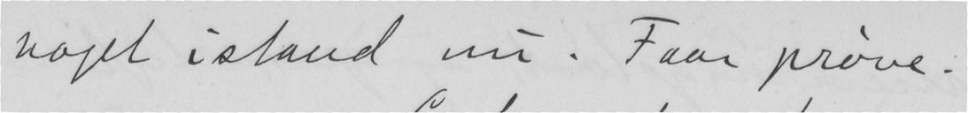noget istand nu. Faar prøve.
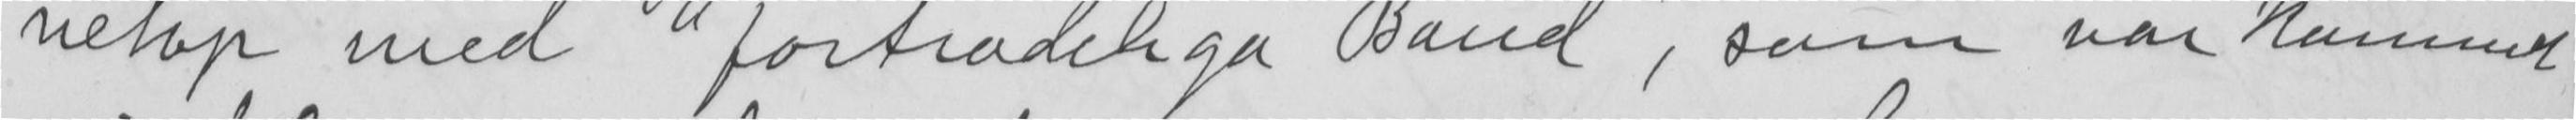netop med "fortrodeliga Band", som var kommet
